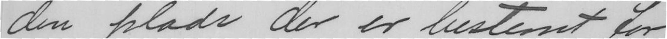den plads der er bestemt for
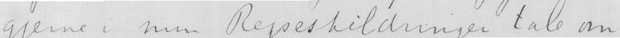gjerne i min Rejseskildringer tale om
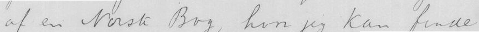af en Norsk Bog, hvor jeg kan finde
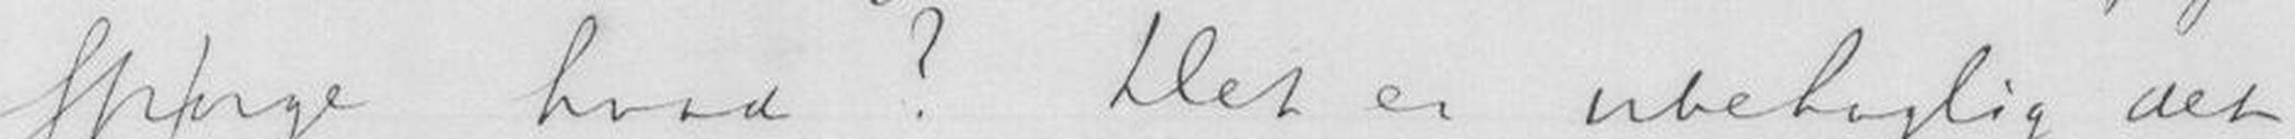spørge hvad? Det er ubehaglig det
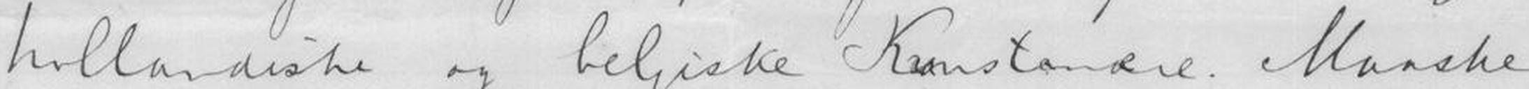hollandiske og belgiske Kunstnære. Maaske
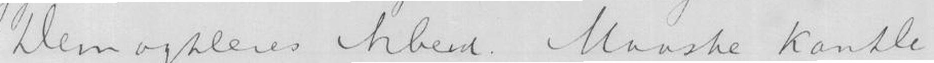Dem og Deres Arbeid. Maaske kan De
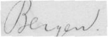Bergen
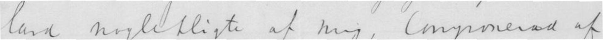land nogle Digte af mig, Componerad af
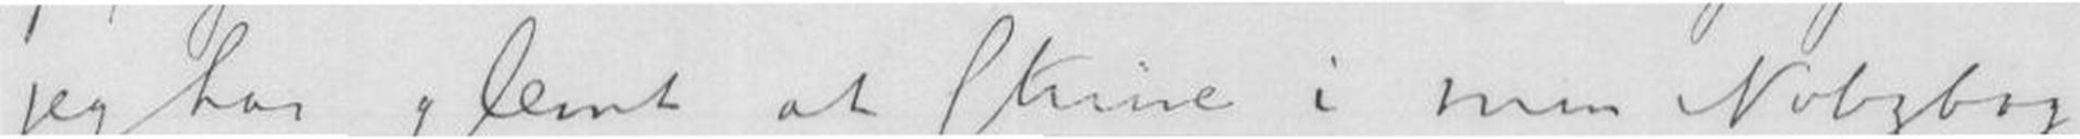jeg har glemt at skrive i min Notizbog
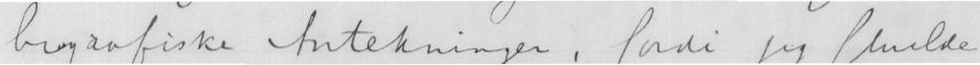biografiske Antekninger, fordi jeg skulde
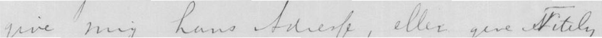give mig hans Adresje, eller give Titelen
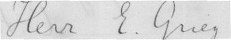Herr E.Grieg
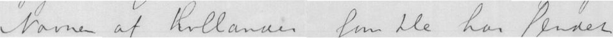Navne af Hollænder som De har sendet
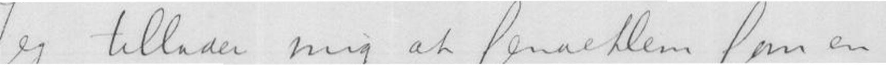Jeg tillader mig at Sende Dem SOM en
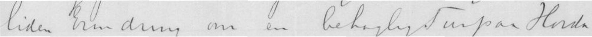liden Erindring om en behaglig Tur paa Horda
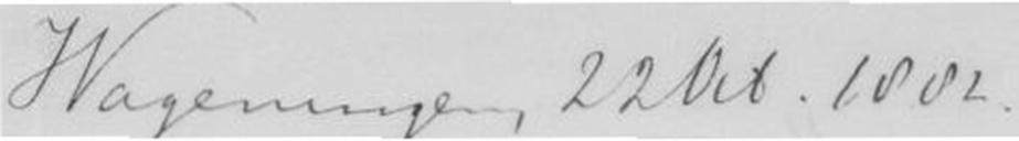Wagening, 22Okt.1882
